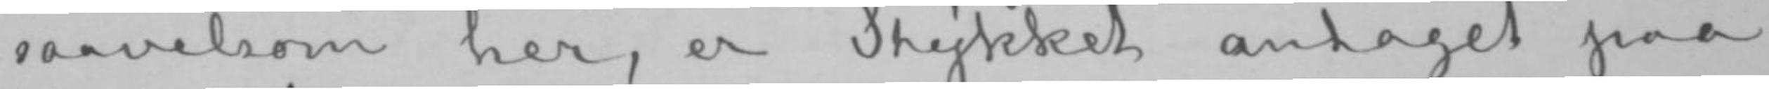saavelsom her, er Stykket antaget paa
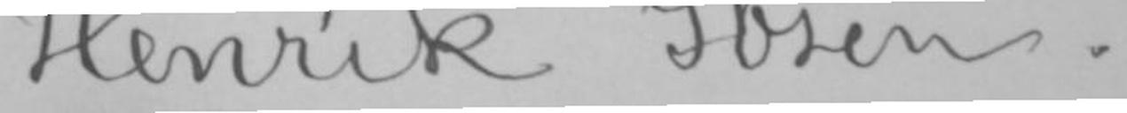Henrik Ibsen.
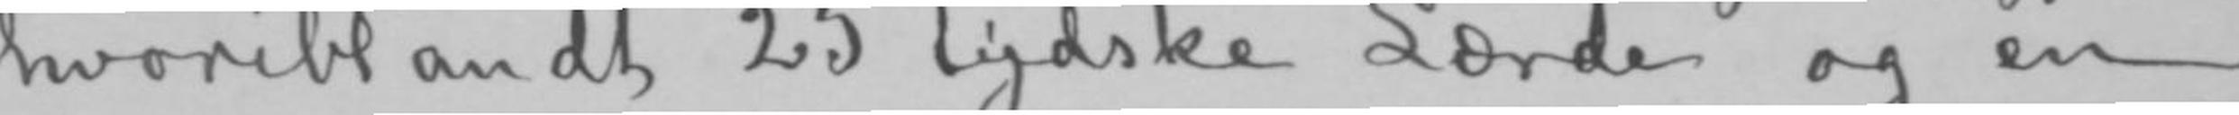hvoriblandt 25 tydske Lærde og en
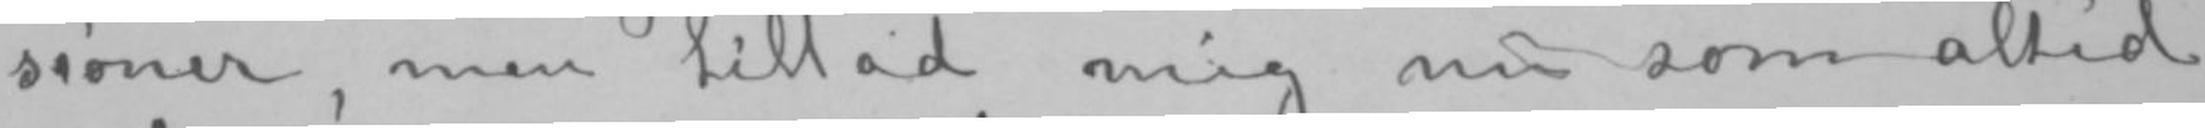sioner, men tillad mig nu som altid
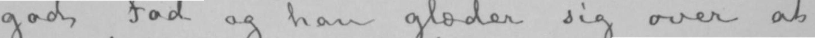god Fod og han glæder sig over at
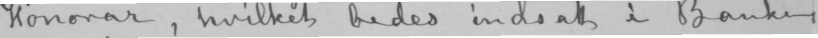Honorar, hvilket bedes indsatt i Banken
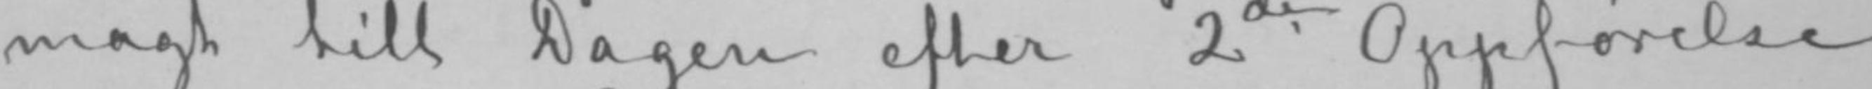magt till Dagen efter 2den Oppførelse
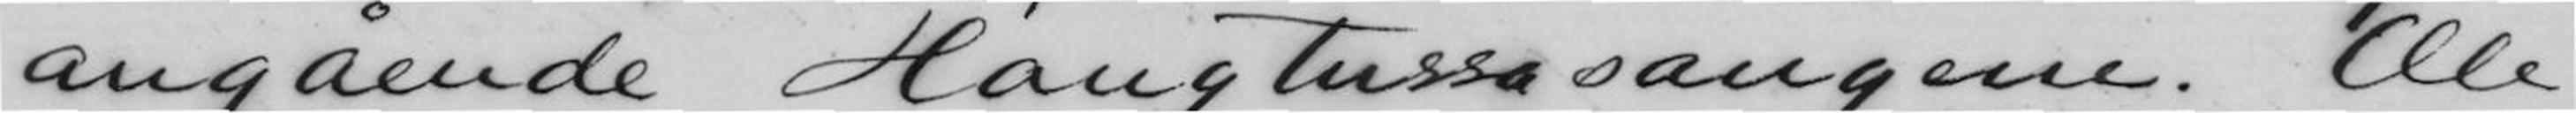angående Haugtussasangene. Ole
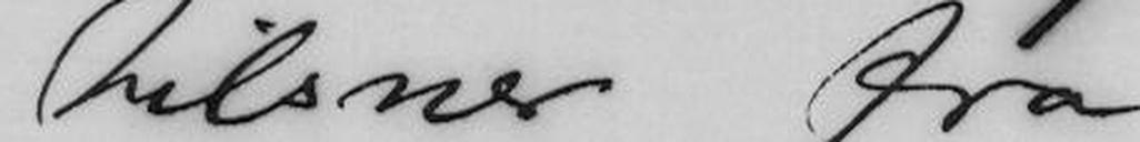hilsner fra
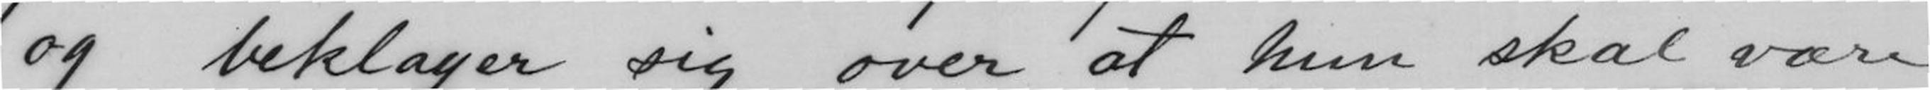og beklager sig over at hun skal være
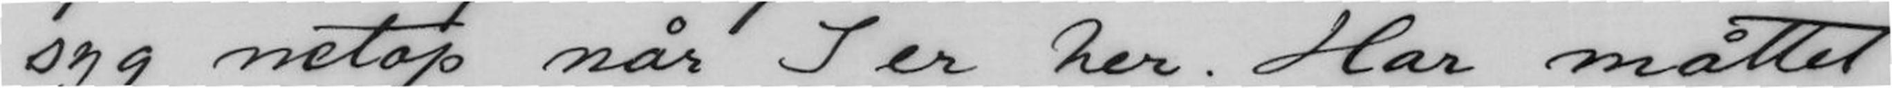syg netop når I er her. Har måttet
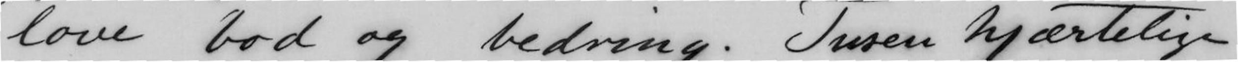love bod og bedring. Tusen hjærtelige
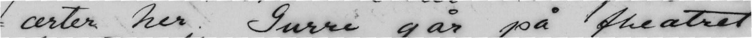=certer her. Gurre går på theatret
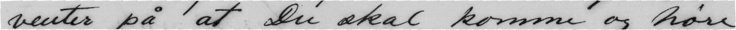venter på at Du skal komme og høre
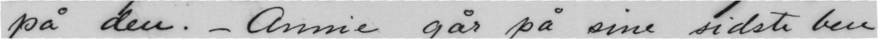på den. - Annie går på sine sidste ben
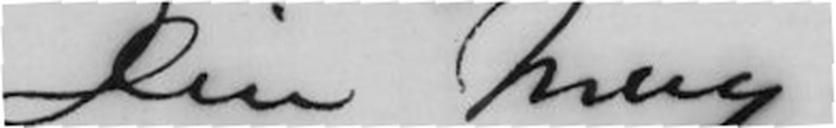Din heng.
